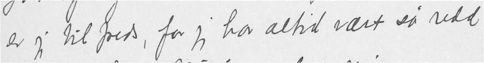er jeg tilfreds, for jeg har altid vært så redd
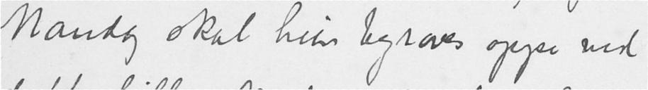Mandag skal hun begraves oppe ved
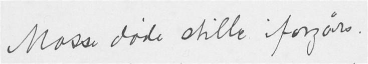Mosse døde stille iforgårs.
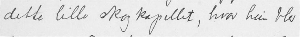dette lille skogkapellet, hvor hun blev
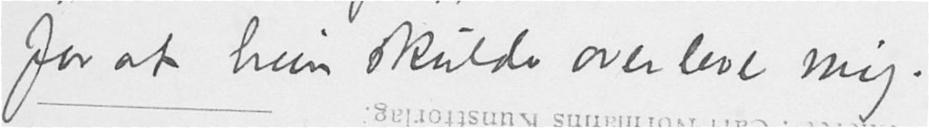for at hun skulde overleve mig.
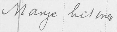Mange hilsener
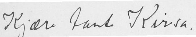Kjære tante Kirsa,
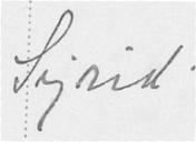Sigrid.
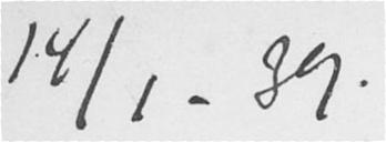14/1-39.
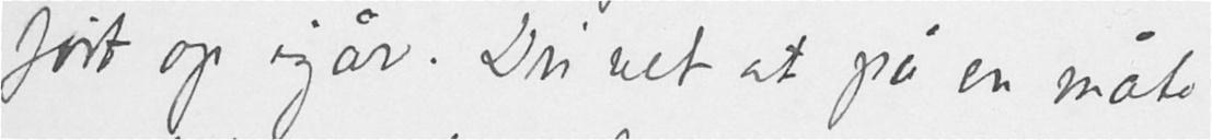ført op igår. Du vet at på en måte
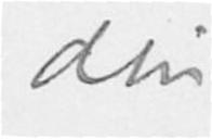din
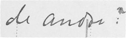de andre?
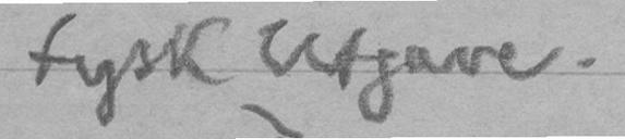tysk Utgave.
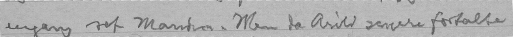engang set Manden. Men da Arild senere fortalte
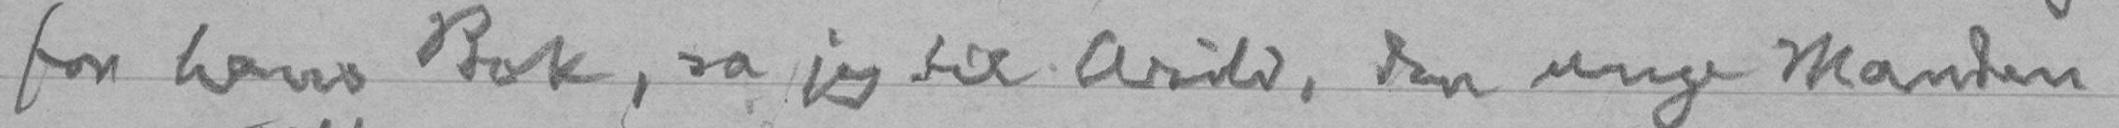for hans Bok, sa jeg til Arild, den unge Manden
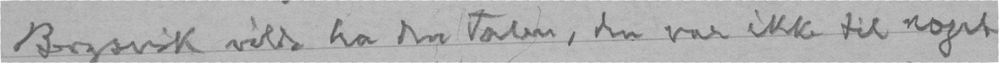Bergsvik vilde ha den Talen, den var ikke til noget
